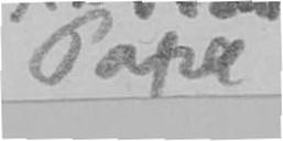Papa
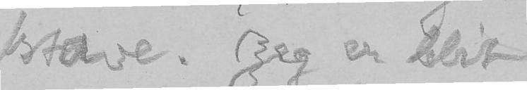stave. Jeg er blit
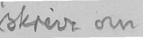skrive om
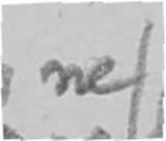ne
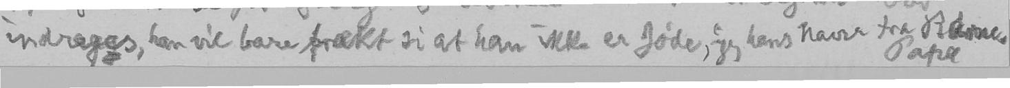indrages, han vil bare frækt si at han ikke er Jøde, jeg hans Navn fra Bladene.
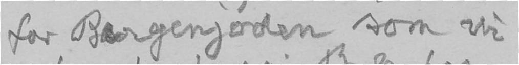for Bergenjøden som vi
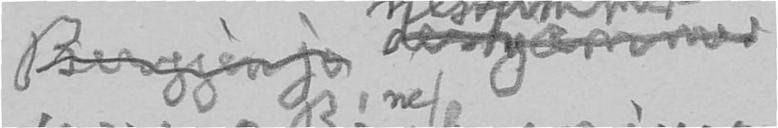Bergjen jo desyemnd
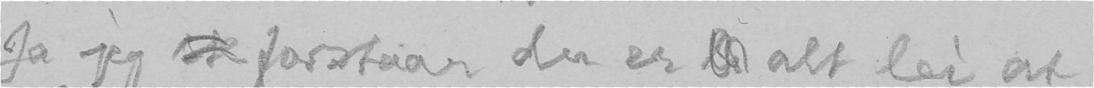Ja jeg sk forstaar du er bi alt lei at
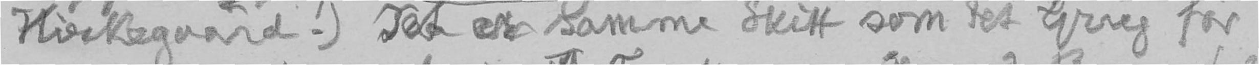Kierkegaard!) Det er samme Skitt som det Grieg før
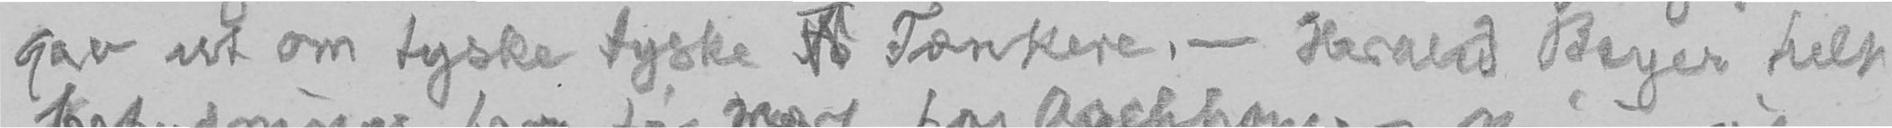gav ut om tyske tyske Fo Tænkere, - Harald Beyer helt
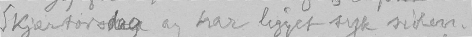Skjærtorsdag og har ligget syk siden.
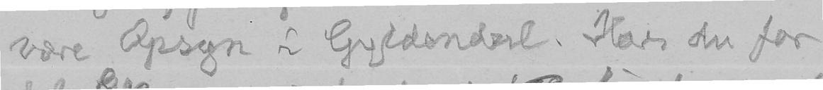være Opsyn i Gyldendal. Har du for
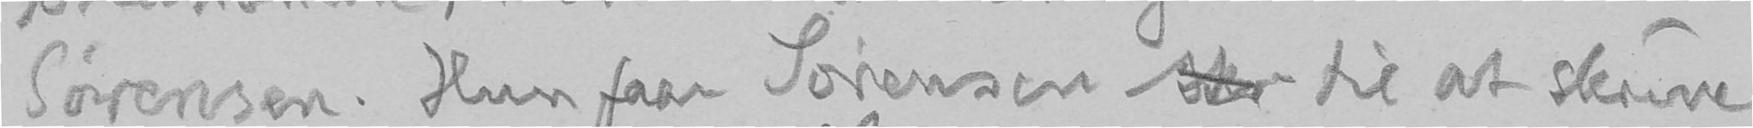Sørensen. Hun faar Sørensen skr til at skrive
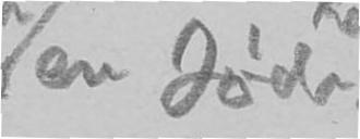en Jøde
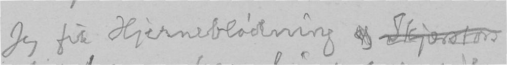Jeg fik Hjerneblødning og Skjærstors
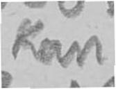kan
